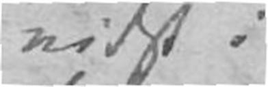vidst i
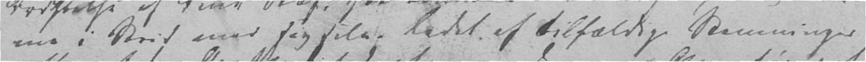me i Strid med sig selv. Ledet af tilfældige Stemninger
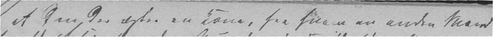at den, der ægter en Kone, fra hvem en anden Mand
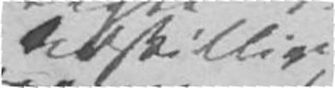adskillige
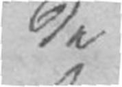de
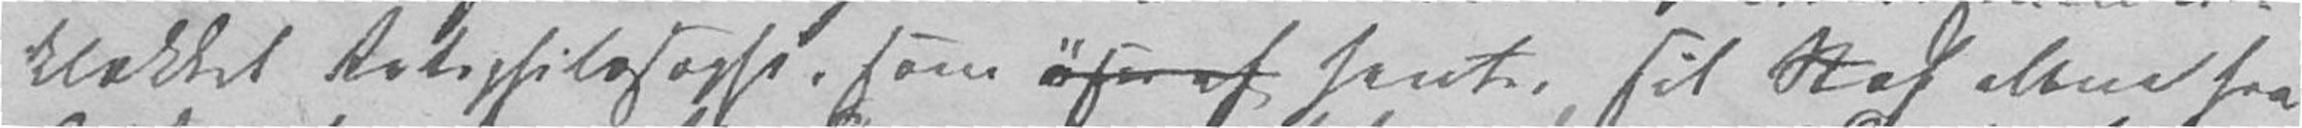klækket Retsphilosophie, som henter sit Stof alene fra
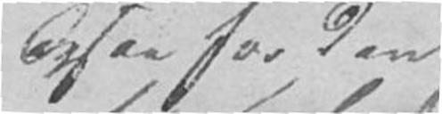Ogsaa for den
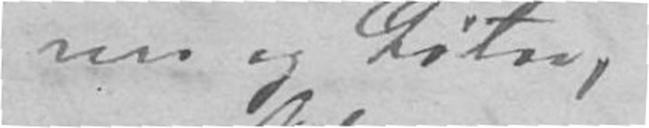ner og Døtre,
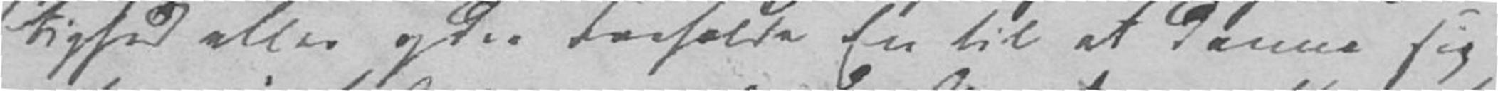tighed eller ydre Forholde En til at danne sig
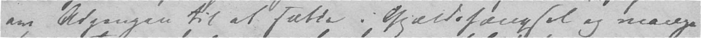om Adgangen til at sætte i Gjældsfængsel og mange
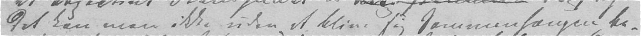det kan man ikke uden at blive sig Sammenhængen be-
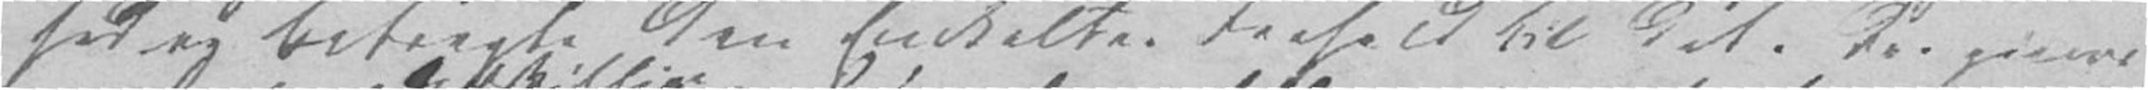hed og betragte den Enkeltes Forhold til det. Der gives
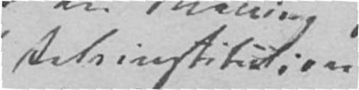Retsinstitution
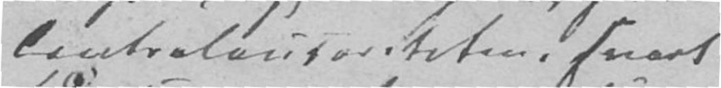Centralautoriteten, snart
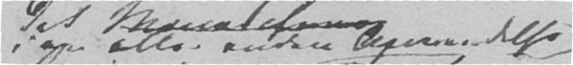i en eller anden Anvendelse
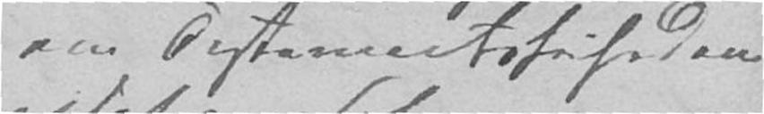om Testamentsfriheden
